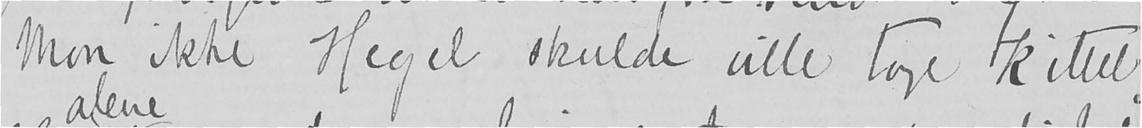Mon ikke Hegel skulde ville tage Kittel-
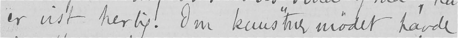er vist herlig. Om kunstnermødet havde
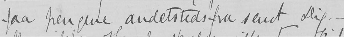faa pengene andetstedsfra sendt Dig. -
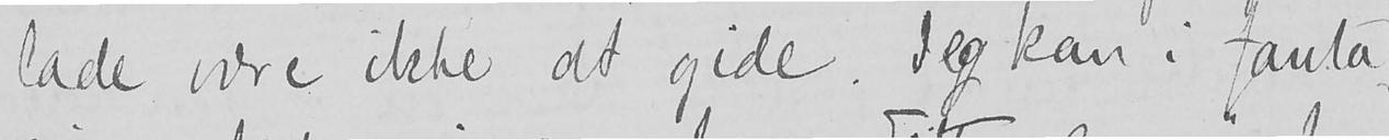lade være ikke at gide. Jeg kan i fanta-
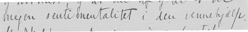megen sentimentalitet i den vennehjælp.
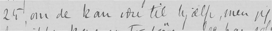25, om de kan være til hjælp, men jeg
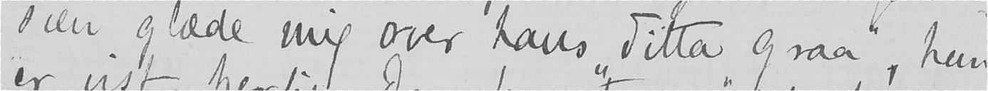sien glæde mig over hans "Titta Graa", hun
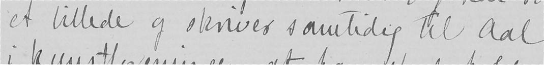et billede og skriver samtidig til Aal
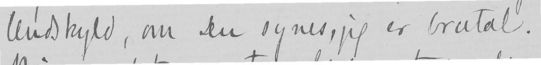Undskyld, om Du synes, jeg er brutal.
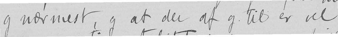og nærmest, og at der af og til er vel
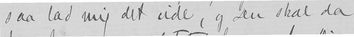saa lad mig det vide, og Du skal da
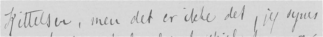Kittelsen, men det er ikke det, jeg synes
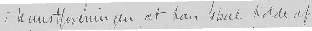i kunstforeningen, at han skal holde af
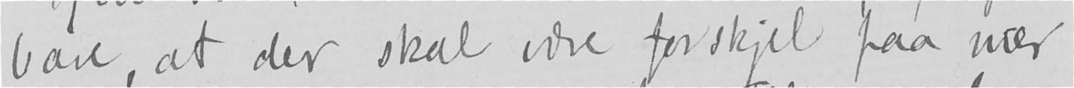bare, at der skal være forskjel paa nær
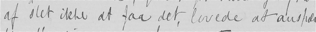af slet ikke at faa det, lovede at anspæn-
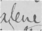alene
